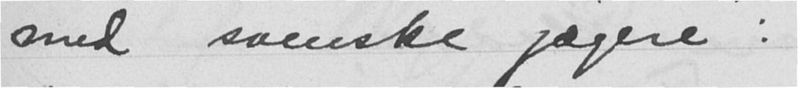med svenske jægere:
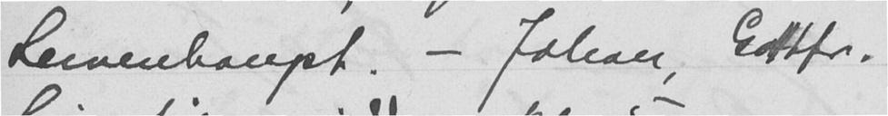Lewenhaupt. - Johan, Gottfr.
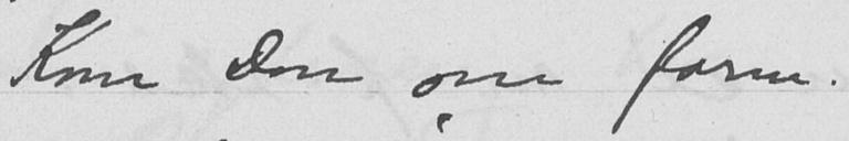Kom Don om form.
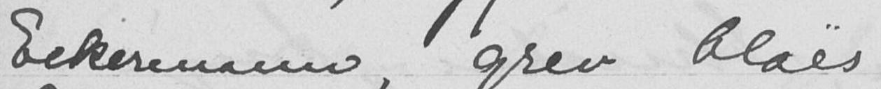Eckermann, grev Claes
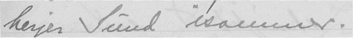herjer Sund isommer.
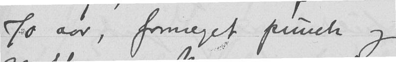70 aar, formeget punch og
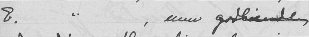E. " , men godbundlig
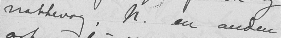nattevog, N. en anden
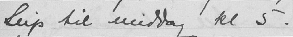Seip til middag kl 5.
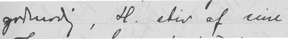godmodig, H. stiv af sine
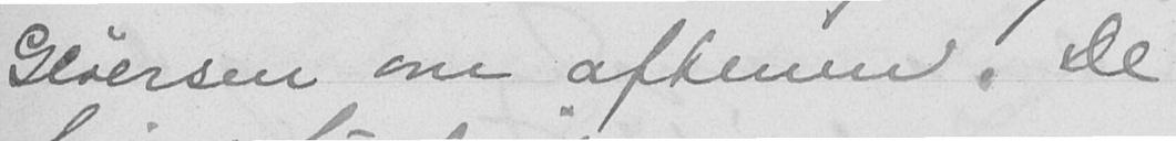Gløersen om aftenen. De
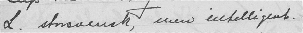L. storsvensk, men intelligent.
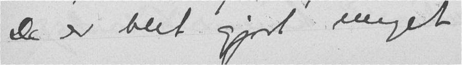De er blit gjort meget
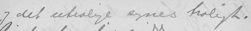og det utrolige synes troligt.
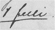1. juli.
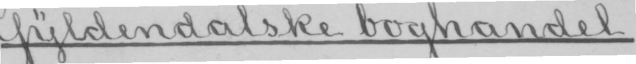Gyldendalske boghandel.
